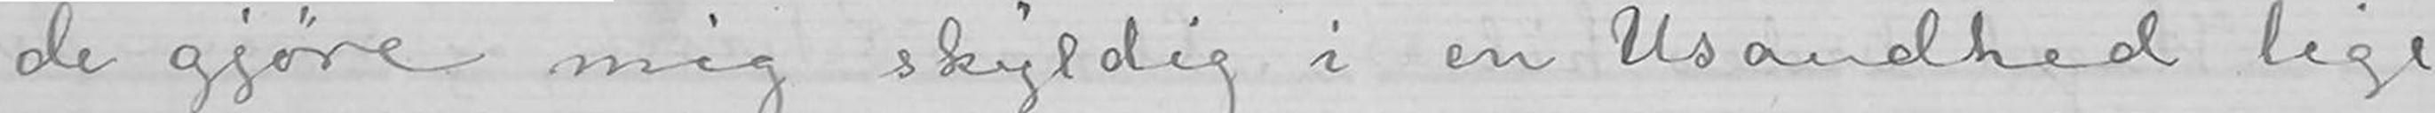de gjøre mig skyldig i en Usandhed lige-
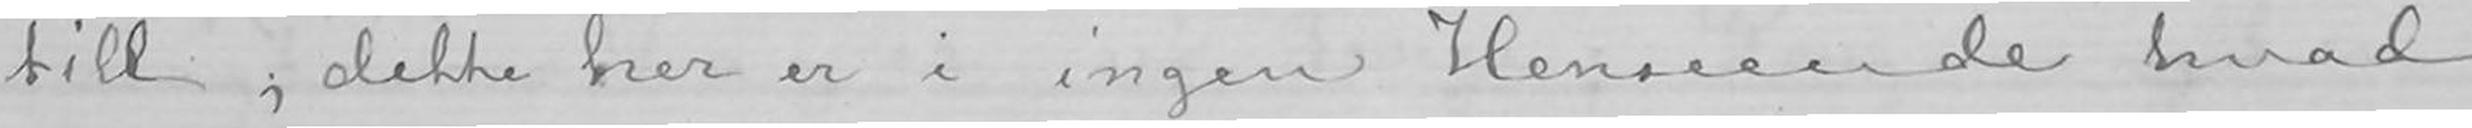till; dette her er i ingen Henseende hvad
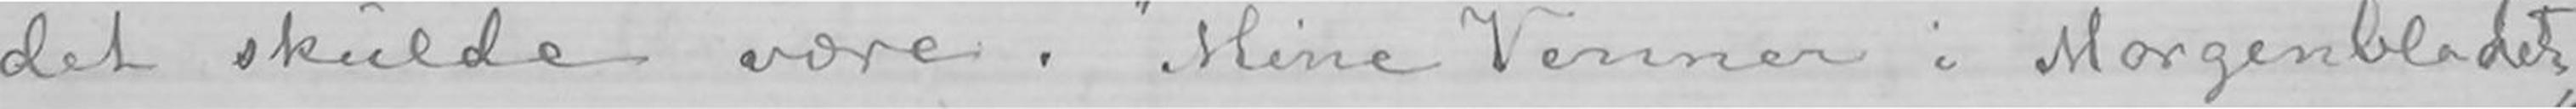det skulde være. «Mine Venner i Morgenbladet»
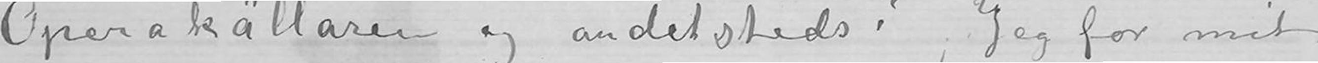Operakällaren og andetsteds? Jeg for mit
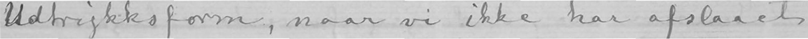Udtrykksform, naar vi ikke har afslaaet
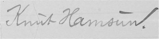Knut Hamsun.
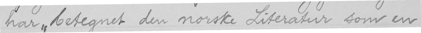har "betegnet den norske Literatur som en
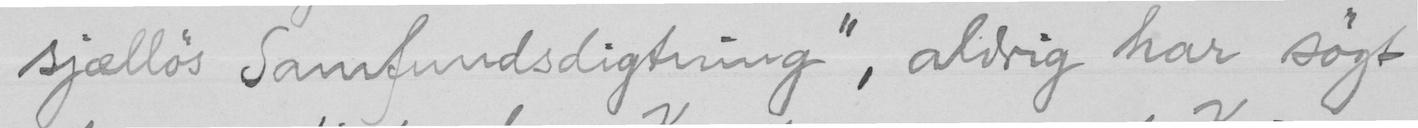sjælløs Samfundsdigtning", aldrig har søgt
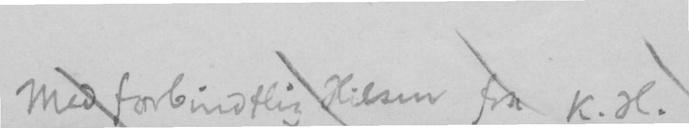Med forbindtlig Hilsen fra K. H.
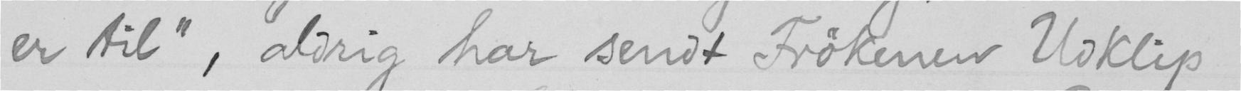er til", aldrig har sendt Frøkenen Udklip
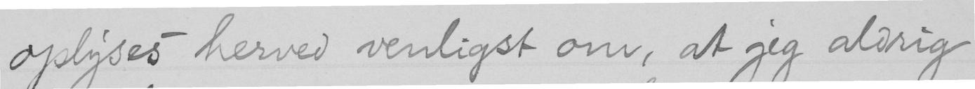oplyses herved venligst om, at jeg aldrig
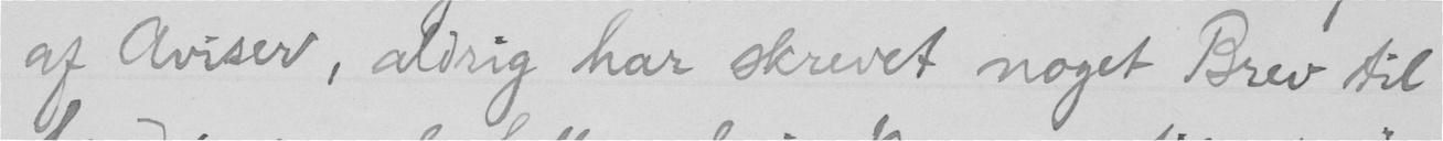af Aviser, aldrig har skrevet noget Brev til
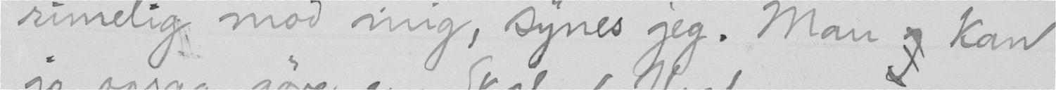rimelig mod mig, synes jeg. Man g kan
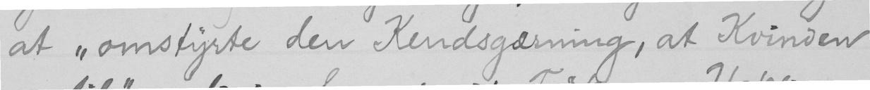at "omstyrte den Kendsgærning, at Kvinden
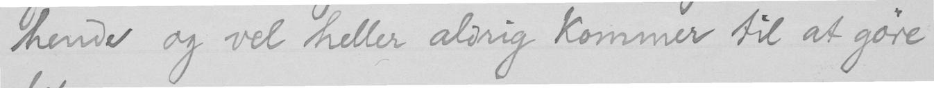hende og vel heller aldrig kommer til at gøre
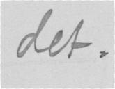det.
